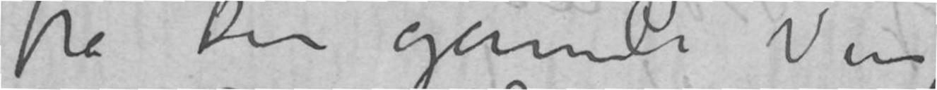fra Din gamle Ven
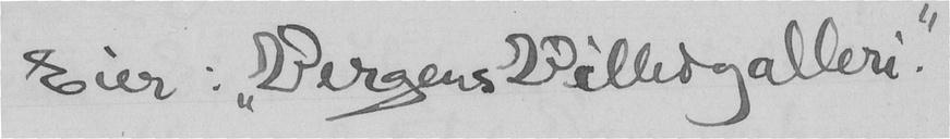Eier: "Bergens Billedgalleri".
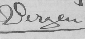Bergen
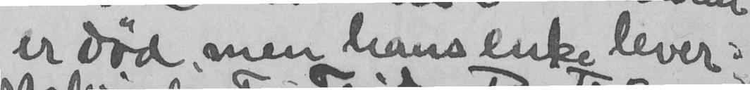er død, men hans enke lever:
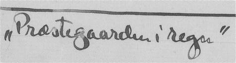"Præstegaarden i regn"
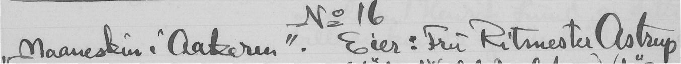"Maaneskin i Aakeren". Eier: Fru Ritmester Astrup
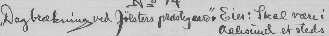"Dagbrækning ved Jølsters præstegaard". Eier: Skal være i
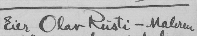Eier Car Rusti - Maleren
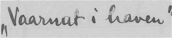"Vaarnat i haven"
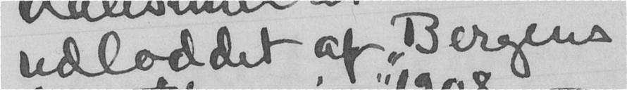udloddet af "Bergens
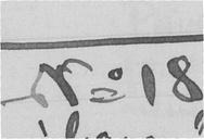No 18
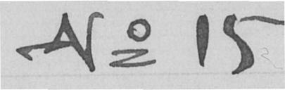No 15
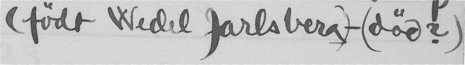(født Wedel Jarlsberg) - (død?)
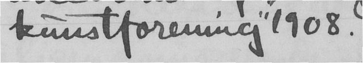kunstførening" 1908.
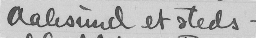Aalesund et steds -
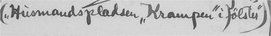("Husmandspladsen "Krampen" i Jølster")
